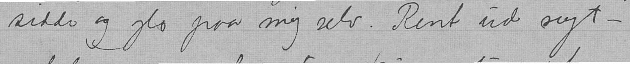sidde og glo paa mig selv. Rent ud sagt -
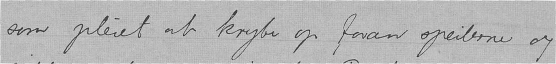som pleiet at krybe op foran speilerne og
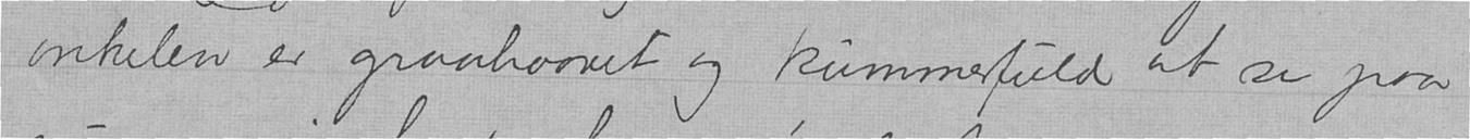onkelen er graahaaret og kummerfuld at se paa
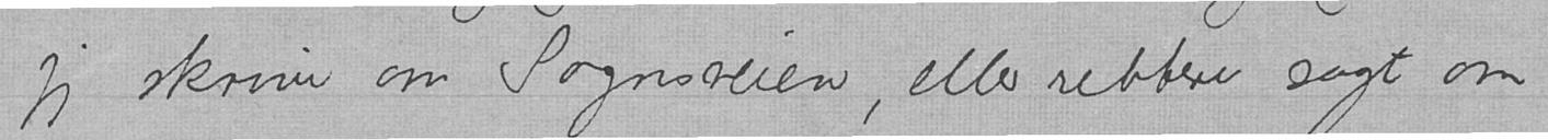jeg skrive om Sognsveien, eller rettere sagt om
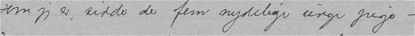som jeg er, sidder der fem nydelige unge pige -
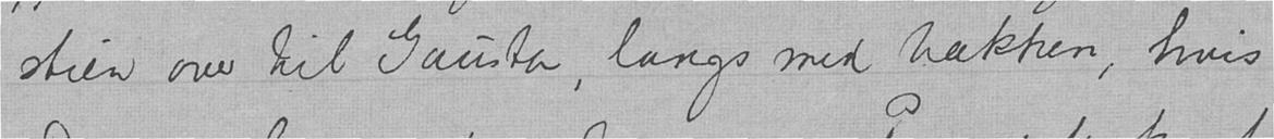stien over til Gausta, langs med bækken, hvis
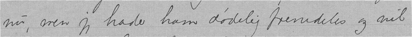nu, men jeg hader ham dødelig fremdeles og vil
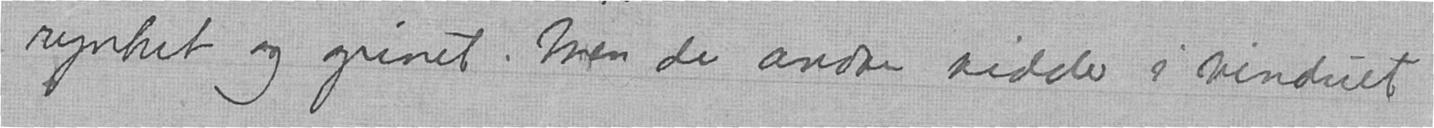rynket og grinet. Men de andre sidder i vinduet
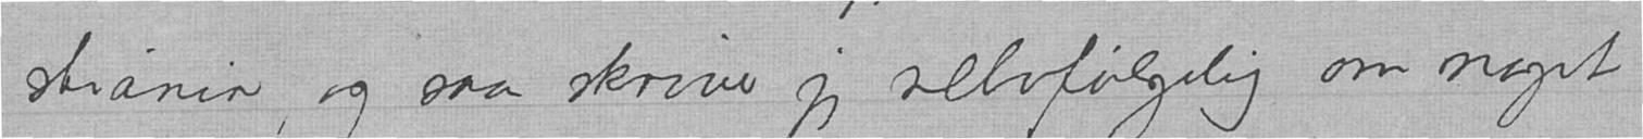stiania, og saa skriver jeg selvfølgelig om noget
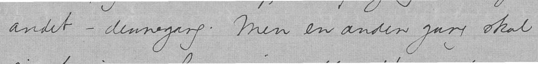andet – dennegang. Men en anden gang skal
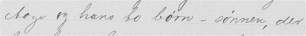Aage og hans to børn – sønnen, der
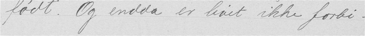født. Og endda er livet ikke forbi -
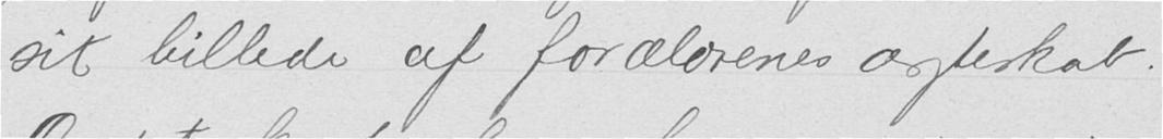sit billede af forældrenes ægteskab.
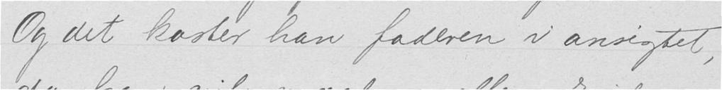Og det kaster han faderen i ansigtet,
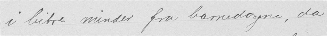i bitre minder fra barnedagene, da
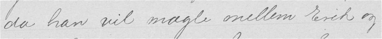da han vil mægle mellem Erik og
